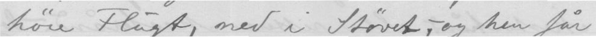høre Flugt, ned i Støvet, og hen får
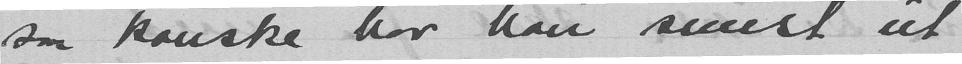saa kanske har han snust ut
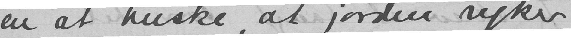en at huske, at jorden ryker
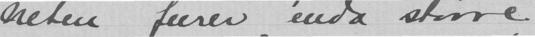heten feirer enda større
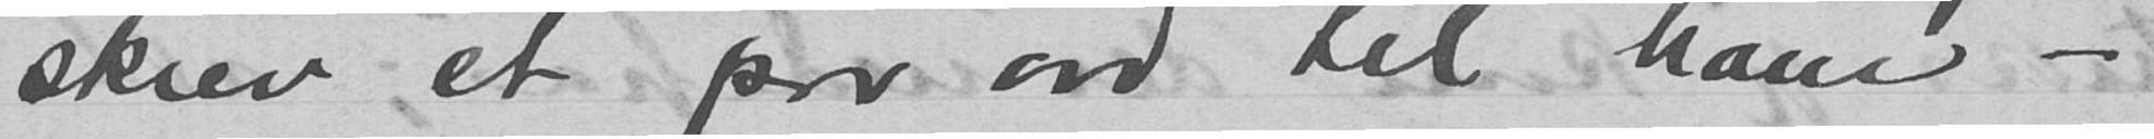skrev et par ord til ham -
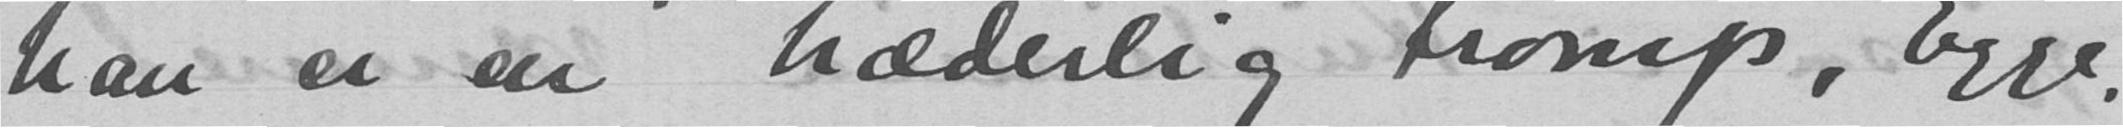han er en hæderlig tromp, Egge,
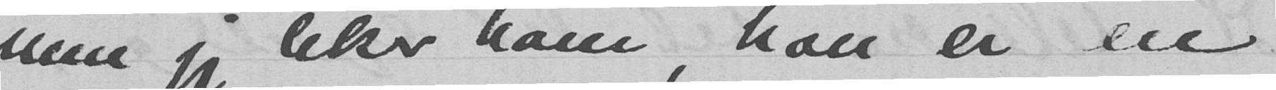men jeg liker ham, han er en
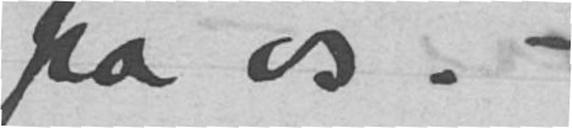fra os. -
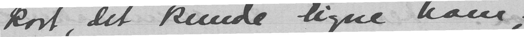kort, det kunde ligne ham;
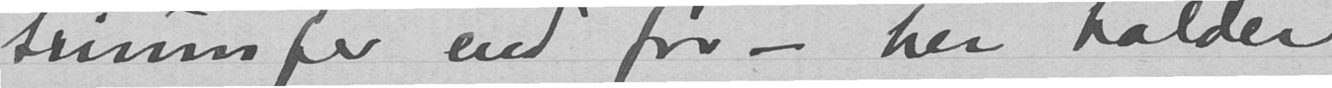triumfer end før - her holder
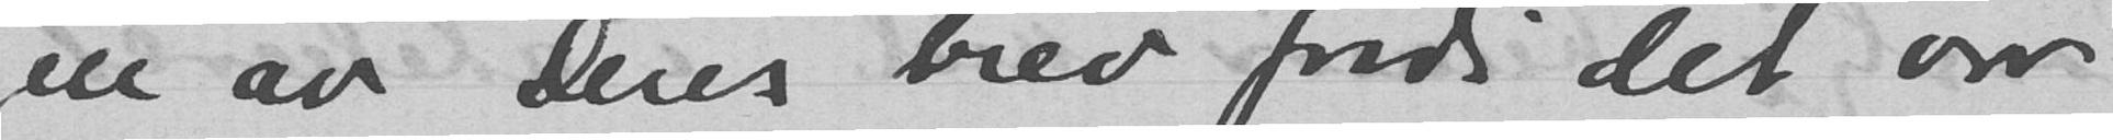en av Deres brev fordi det var
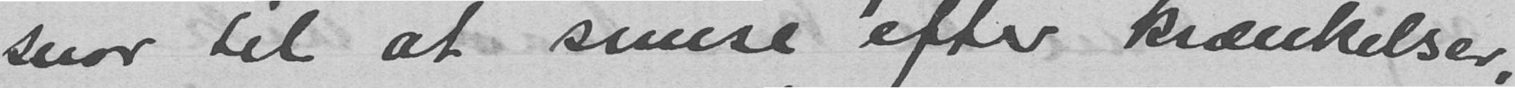snar til at snuse efter krænkelser,
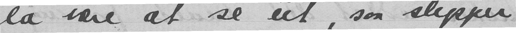la vere at se ut, saa slipper
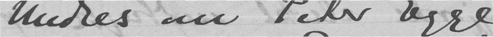Undres om Peter Egge
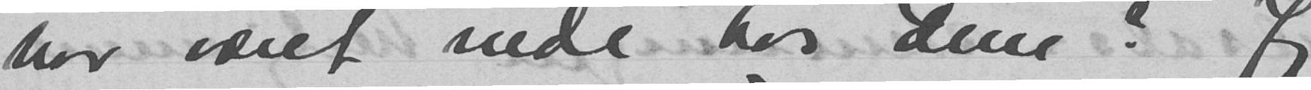har veret nede hos Dem? Jeg
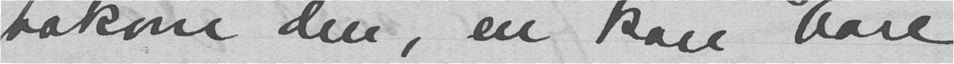bakom den, en kan bare
